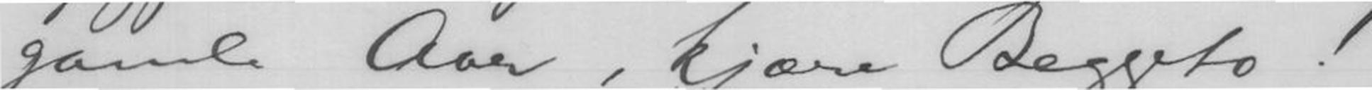gamle Aar, kjære Beggeto!
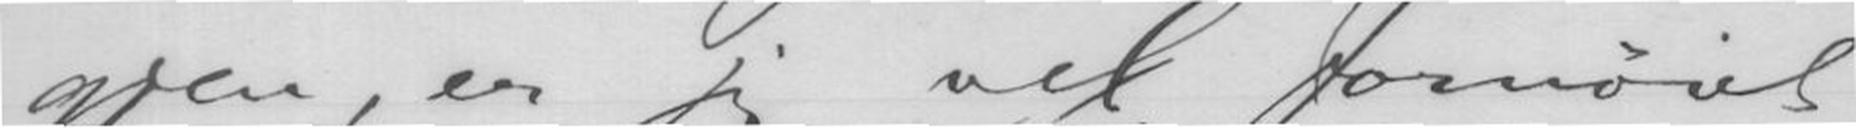gjen, er jeg vel fornøiet
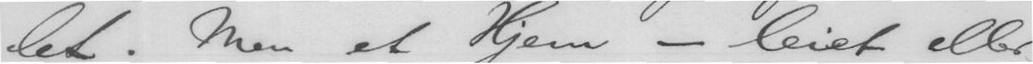let. Men et Hjem - leiet eller
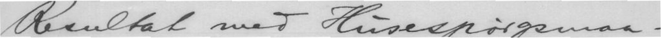Resultat med Husespørgsmaa-
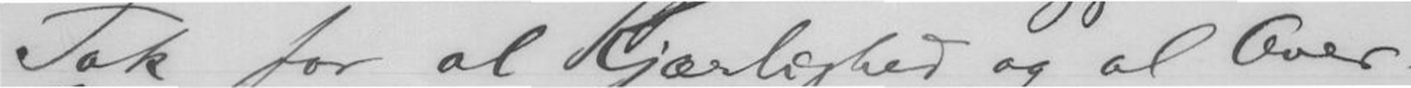Tak for al Kjærlighed og al Over-
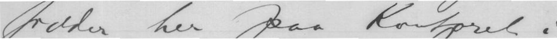sidder her paa Kontoret i-
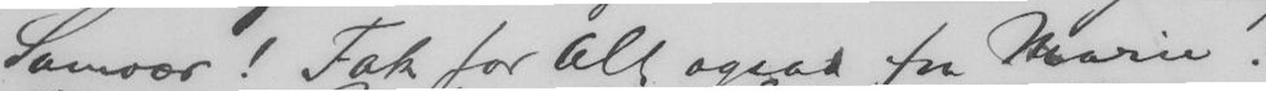Samvær! Tak for Alt ogsaa fra Marie!
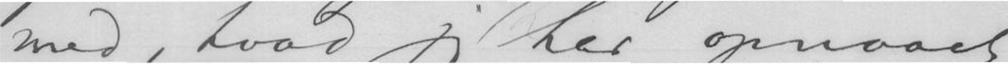med, hvad jeg her opnaaet
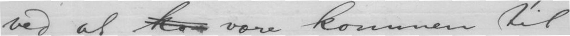ved at kom være kommen til
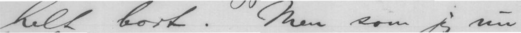helt bort. Men som jeg nu
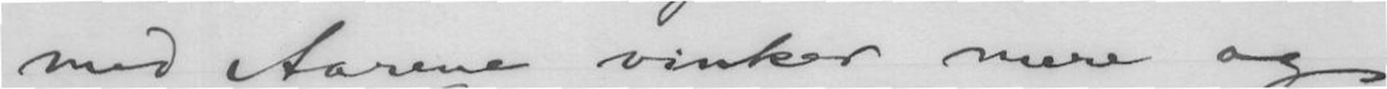med Aarene vinker mere og
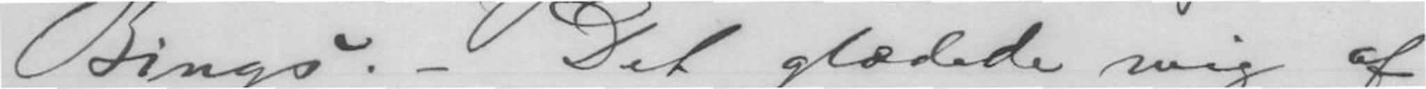Bings. - Det glædede mig af
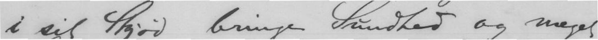i sit Skjød bringe Sundhed og meget
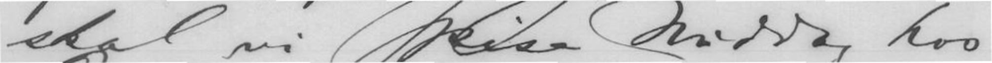skal vi spise Middag hos
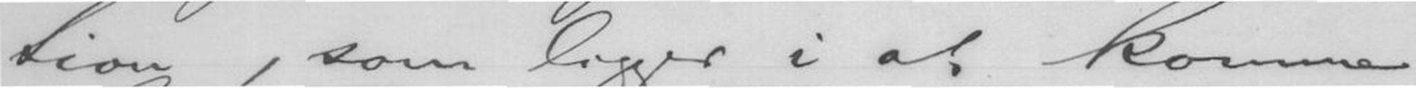tion, som ligger i at komme
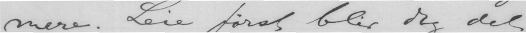mere. Leie først blir dog det
